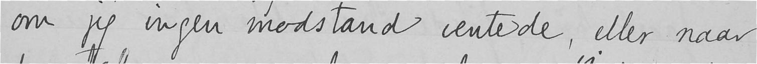om jeg ingen modstand ventede, eller naar
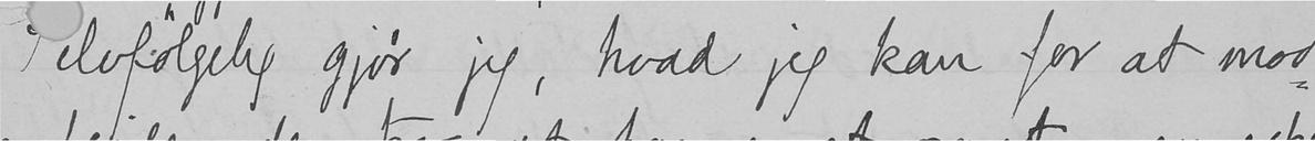Selvfølgelig gjør jeg, hvad jeg kan for at mod-
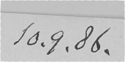10.9.86.
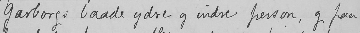Garborgs baade ydre og indre person, og paa
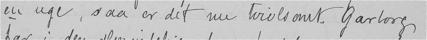en uge, saa er det nu tvivlsomt. Garborg
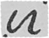vi
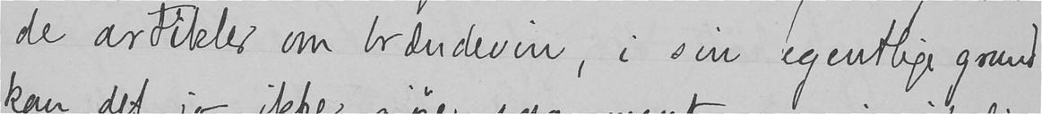de artikler om brændevin, i sin egentlige grund
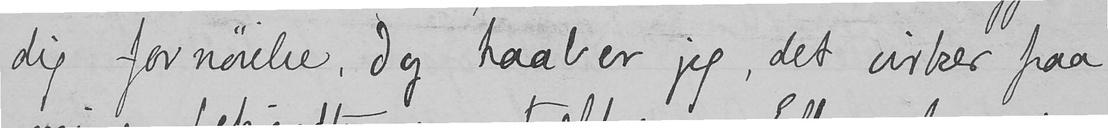dig fornøielse. Dog haaber jeg, det virker paa
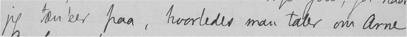jeg tænker paa, hvorledes man taler om Arne
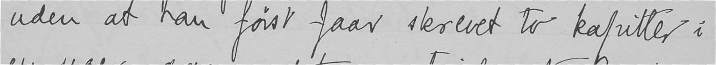uden at han først faar skrevet to kapitler i
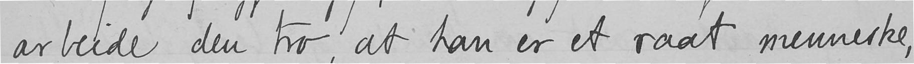arbeide den tro, at han er et raat menneske,
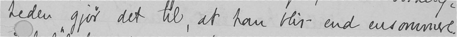heden gjør det til, at han blir end ensommere.
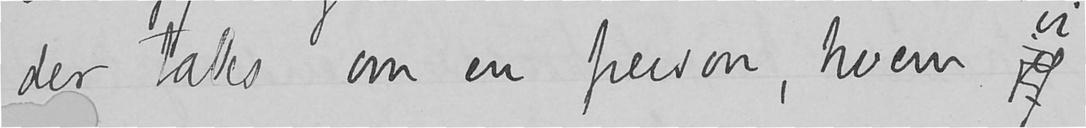der tales om en person, hvem jeg
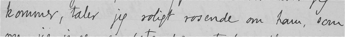kommer, taler jeg roligt rosende om ham, som
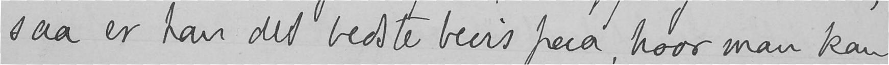saa er han det bedste bevis paa, hvor man kan
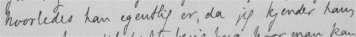hvorledes han egentlig er, da jeg kjender ham,
